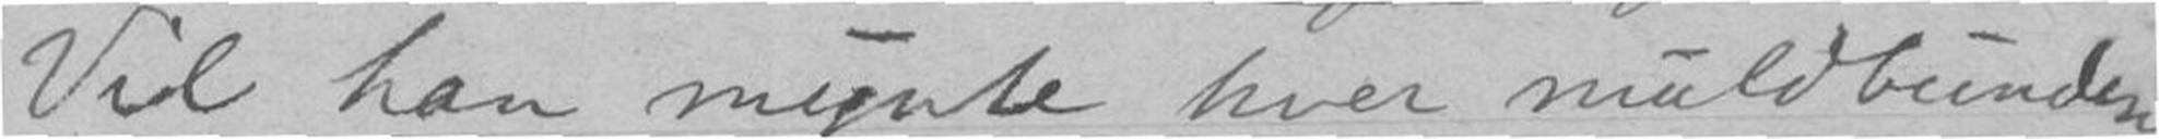Vil han mynte hver muldbunden
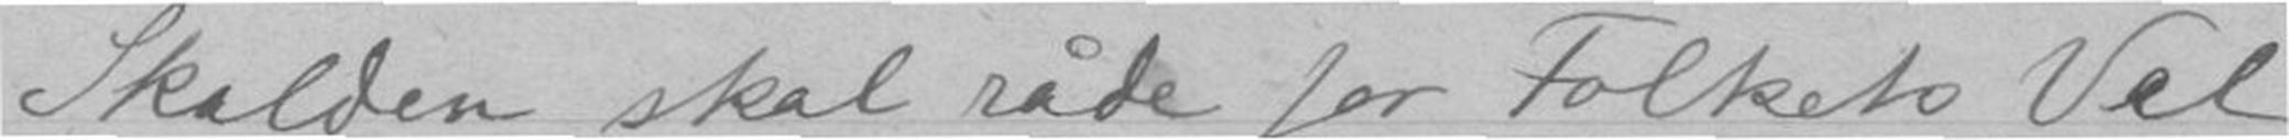Skalden skal råde for Folkets Vel
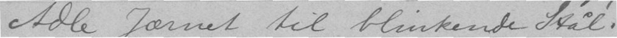Adle Jærnet til blinkende Stål.
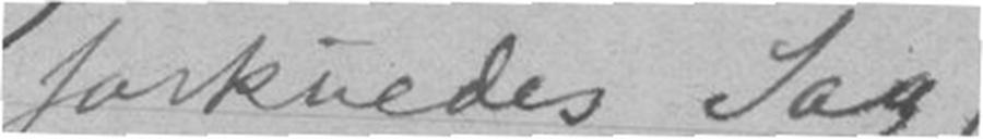forkuedes Sag,
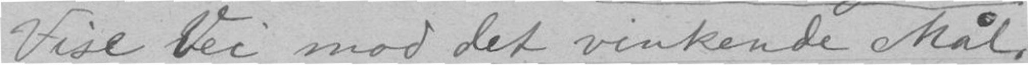Vise Vei mod det vinkende Mål
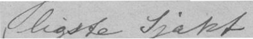ligste Sjakt
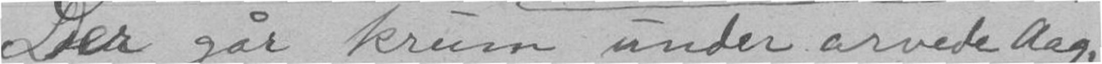Der går krum under arvede Aag.
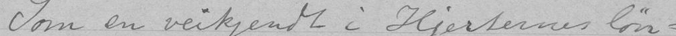Som en veikjendt i Hjerternes løn-
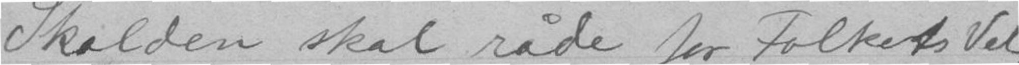Skalden skal råde for Folkets Vel!
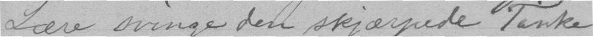Lære svinge den skjærpede Tanke
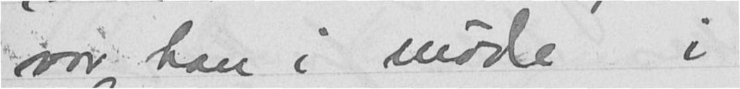var han i møde i
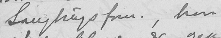Saugbrugsforn., hvor
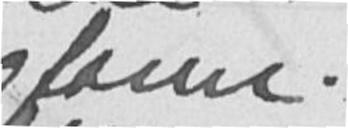form.
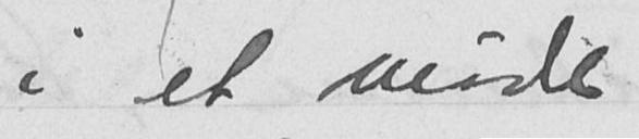i et møde
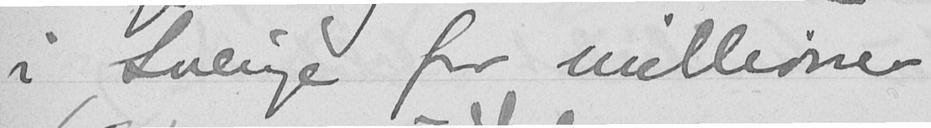i Sverige for millioner
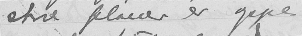store planer er oppe
